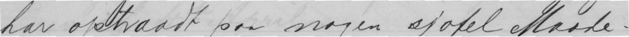har optraadt paa nogen sjofel Maade.
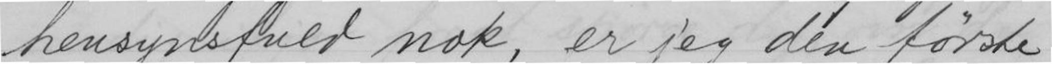hensynsfuld nok, er jeg den første
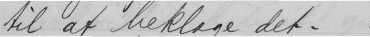til at beklage det.
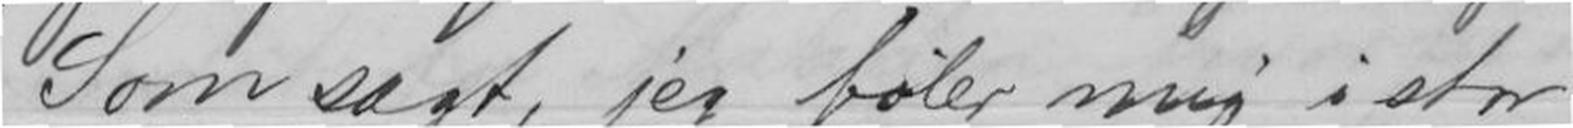Som sagt, jeg føler mig i stor
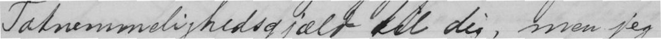Taknemmelighedsgjæld til dig, men jeg
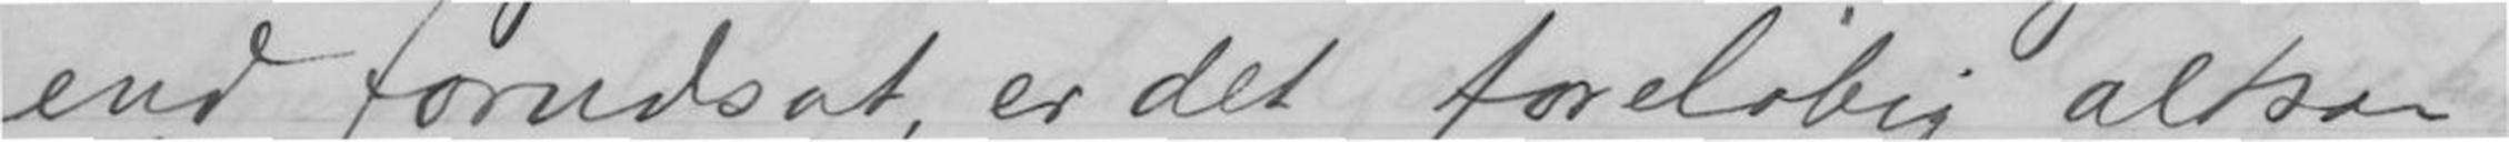end forudsagt, er det foreløbig altsaa
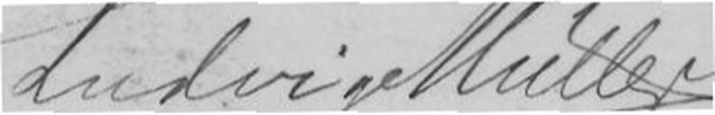Ludvig Müller
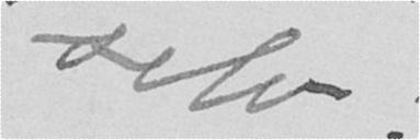selv!
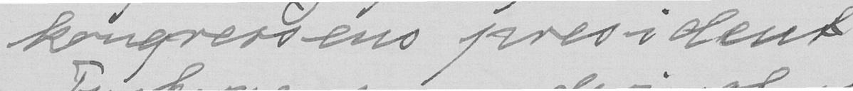kongressens president
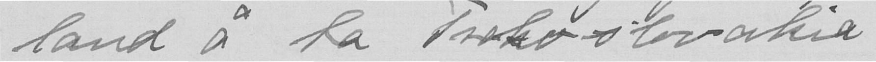land å ta Tsekoslovakia
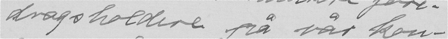dragsholdere på vår kon-
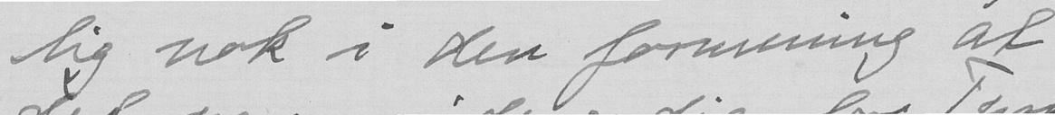lig nok i den formening at
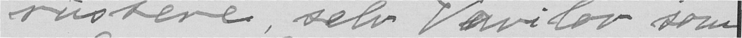russere, selv Vavilov som
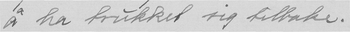å ha trukket sig tilbake.
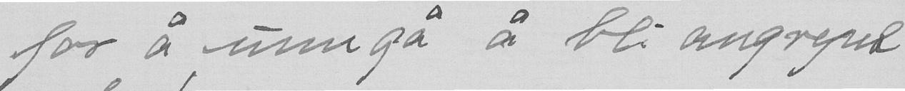for å unngå å bli angrepet
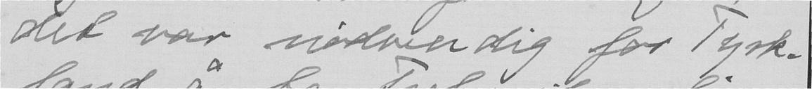det var nødvendig for Tysk-
